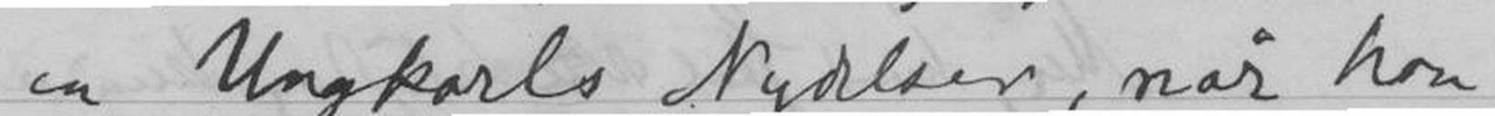en Ungkarls Nydelser, når han
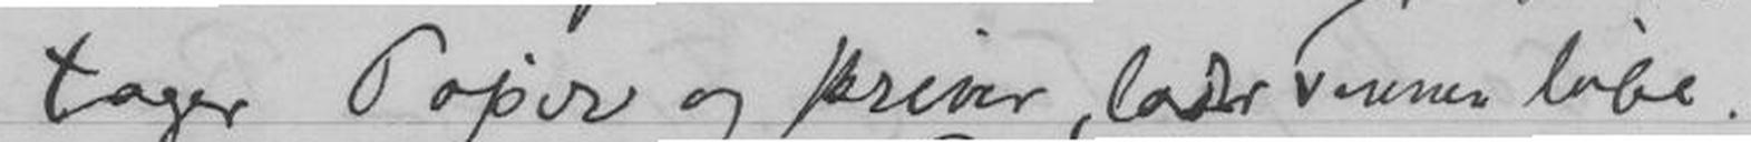tager Papir og Skriver, lader Pennen Løbe.
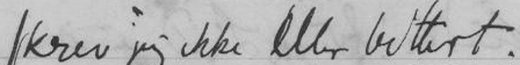skrev jeg ikke heller bittert.
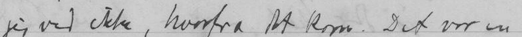jeg ved ikke, hvorfra det kom. Det var en
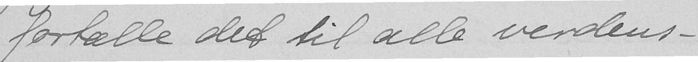fortælle det til alle verdens-
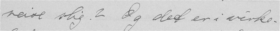reise slig? Og det er i virke-
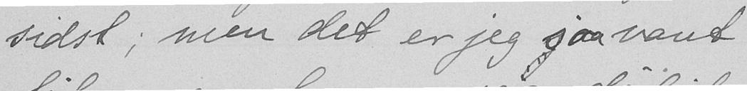sidst; men det er jeg saa vant
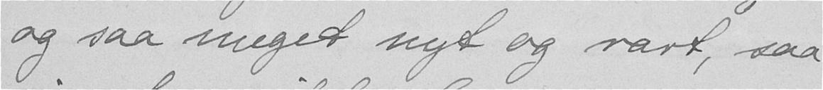og saa meget nyt og rart, saa
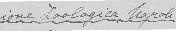Stazione zoologica, Napoli
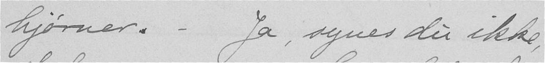hjørner. Ja, synes du ikke
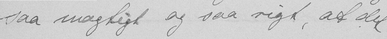saa mægtigt og saa rigt at det
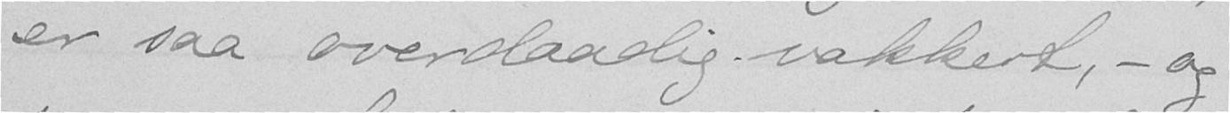er saa overdaadig vakkert, - og
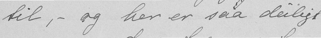til,- og her er saa deiligt
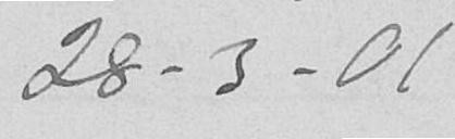28-3-01
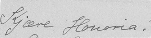Kjære Honoria!
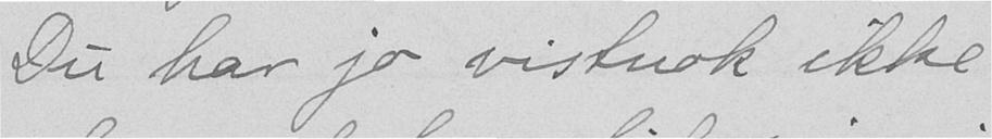Du har jo vistnok ikke
